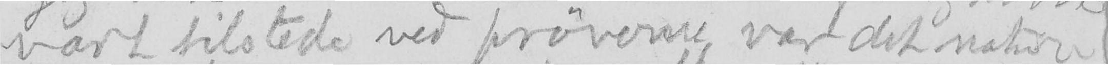vært tilstede ved prøverne var det natur-
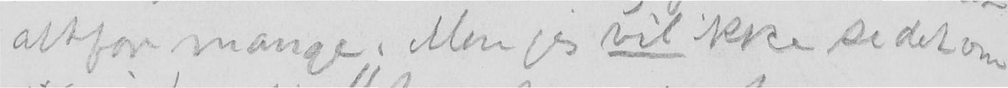altfor mange. Men jeg vil ikke se det om
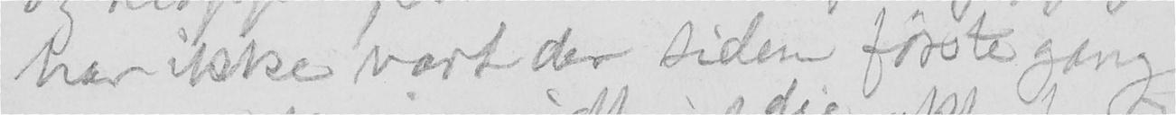har ikke vært der siden første gang
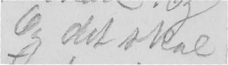Og det skal
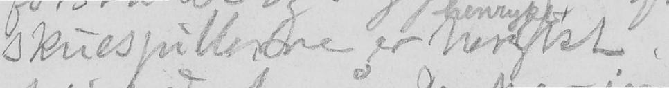skuespillerne er herykt
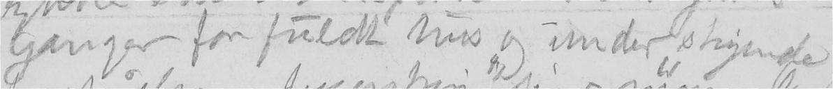ganger for fuldt hus og under "stigende
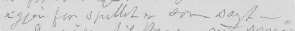igjen for spillet er som sagt -.
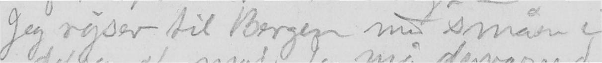Jeg rejser til Bergen med småen i
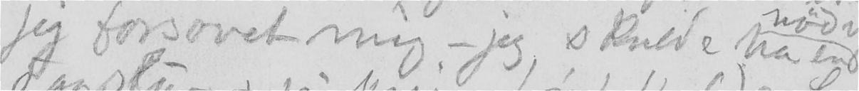jeg forsovet mig - jeg skulde ha en
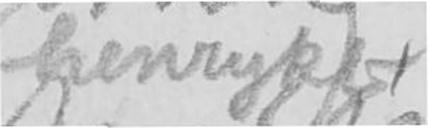henrykt.
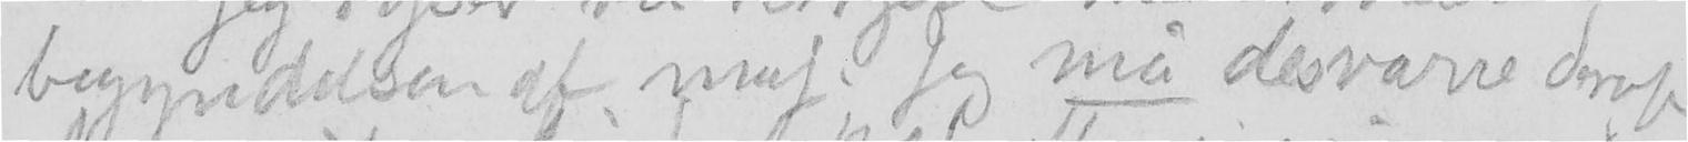begyndelsen af maj. Jeg må desværre derop
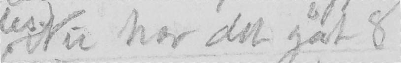Nu har det gåt 8
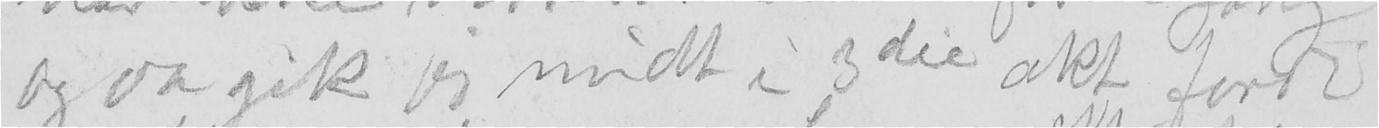og da gik jeg midt i 3die akt fordi
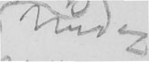mid-
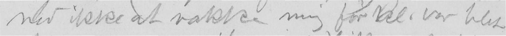ned ikke at vække mig før kl. var blit
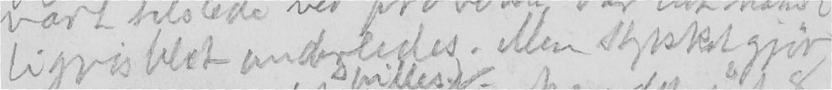ligvis blit anderledes. Men stykket gjør
